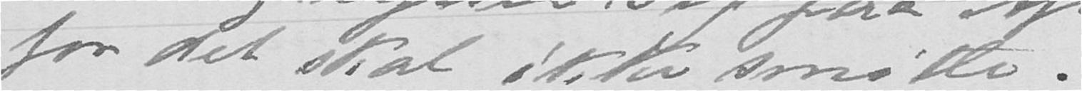for det skal ikke smitte.
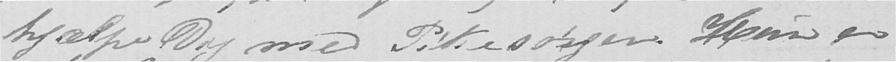hjælpe Dig med Pikesorgen. Hun er
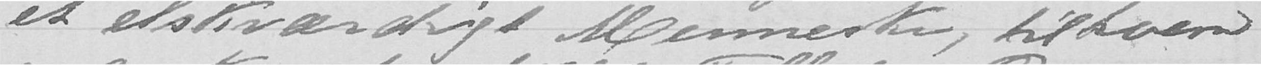et elskværdigt Menneske, tilhvem
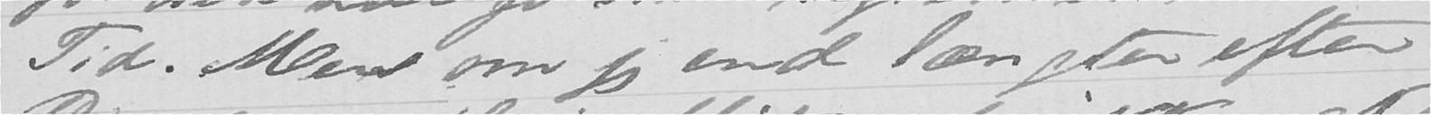Tid. Men om jeg end længter efter
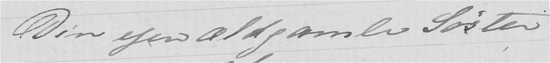Din egen ældgamle Søster
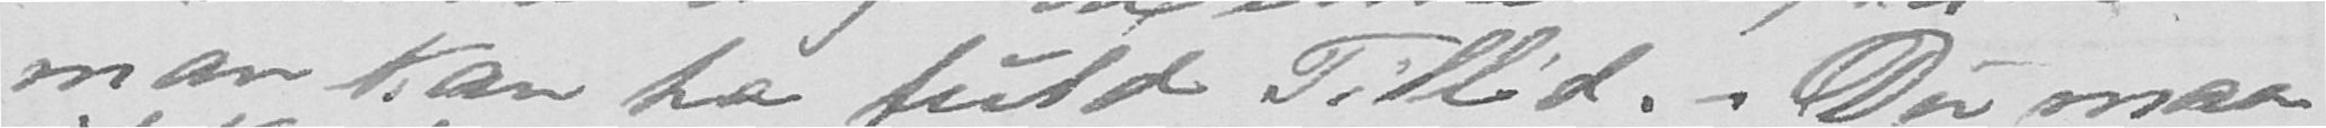man kan ha fuld Tillid. Du maa
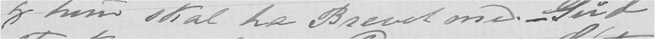og hun skal ha Brevet med. - Gud
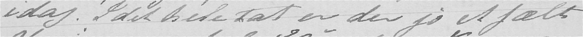idag. Idet hele tat er der jo et fælt
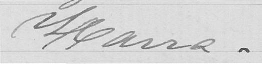Harra.
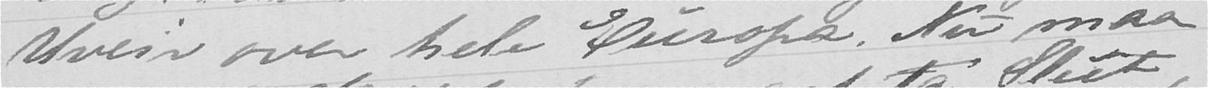Uveir over hele Europa. Nu maa
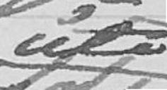ute
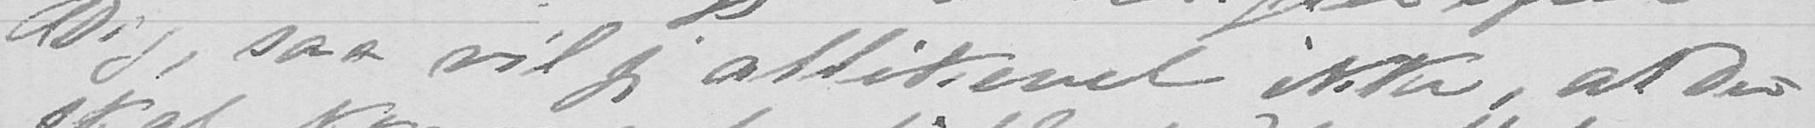Dig, saa vil jeg allikevet ikke, at du
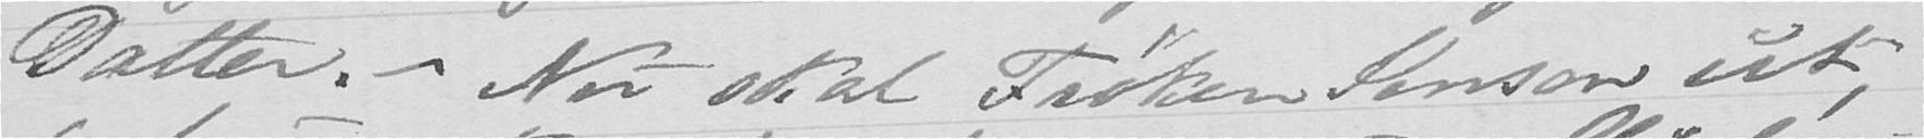Datter. Nu skal Frøken Jonsen ut,
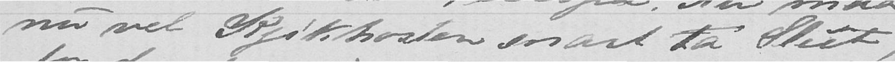nu vel Kjikhosten snart ta Slut
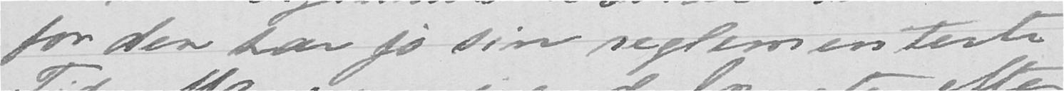for den har jo sin reglementerte
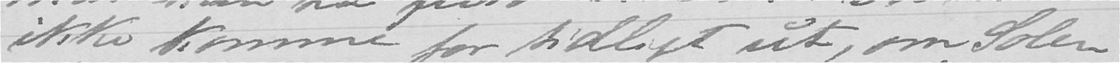ikke komme for tidligt ut, om Solen
